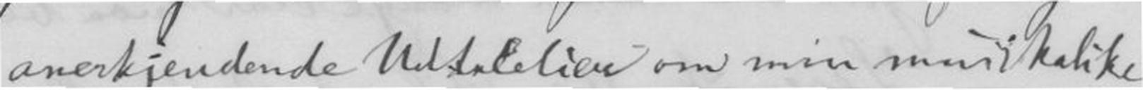anerkjendende Udtalelser om min musikalske
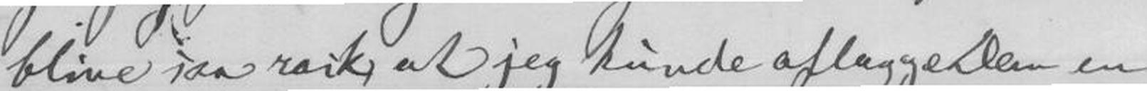blive saa rask, at jeg kunde aflægge Dem en
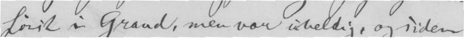først i Grand, men var uheldig, og siden
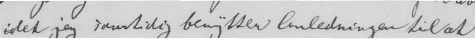idet jeg samtidig benytter Anledningen til at
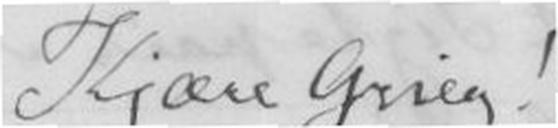Kjære Grieg!
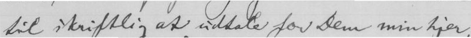til skriftlig at udtale for Dem min hjer-
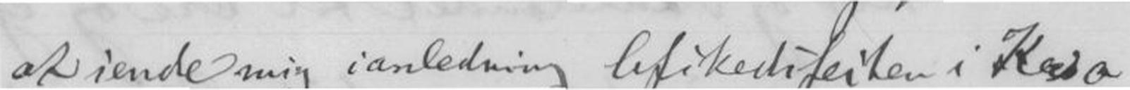at sende mig ianledning Afskedfesten i Kra
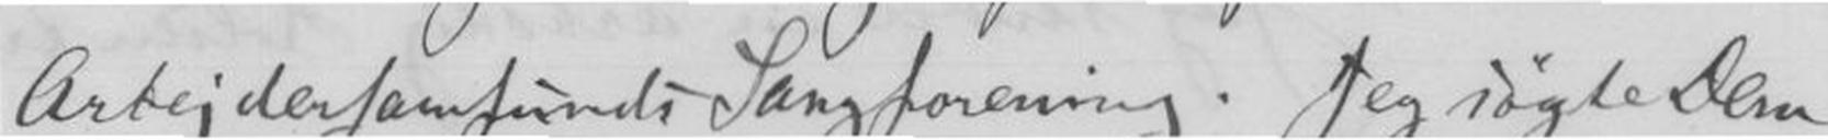Arbejdersamfunds Sangforening. Jeg søgte Dem
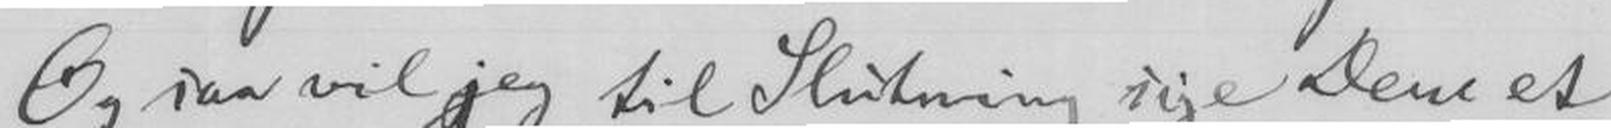Og saa vil jeg til Slutning sige Dem et
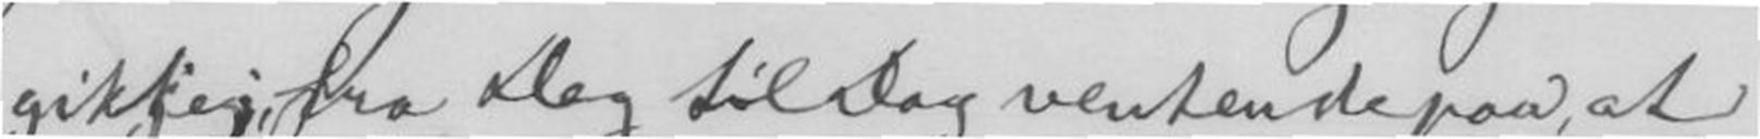gik jeg fra Dag til Dag ventende paa, at
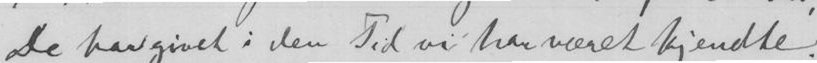De har givet i den Tid vi har været kjendte.
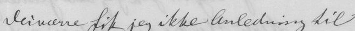Desværre fik jeg ikke Anledning til
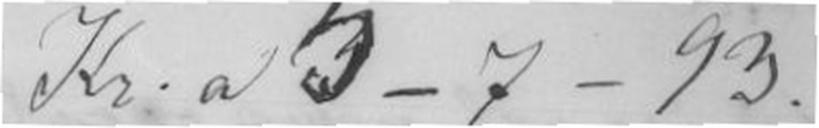Kr.a 5-7-93.
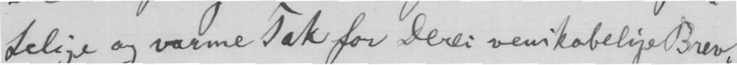telige og varme Tak for Deres Venskabelige Brev,
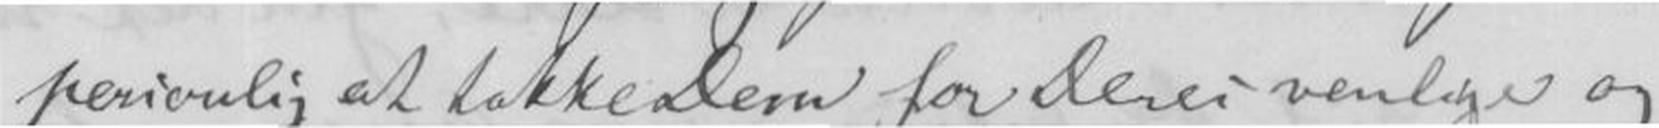personlig at takke Dem for Deres venlige og
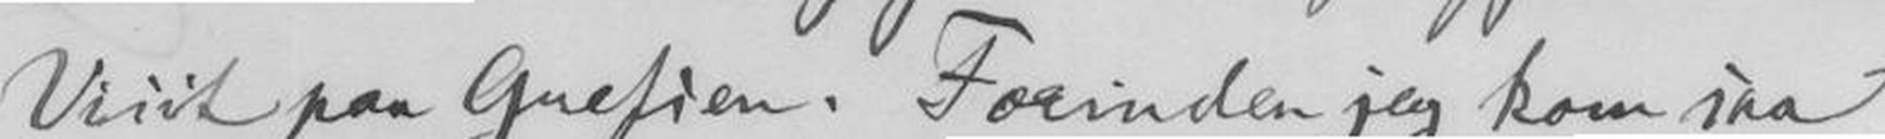Visit paa Grefsen. Forinden jeg kom saa
